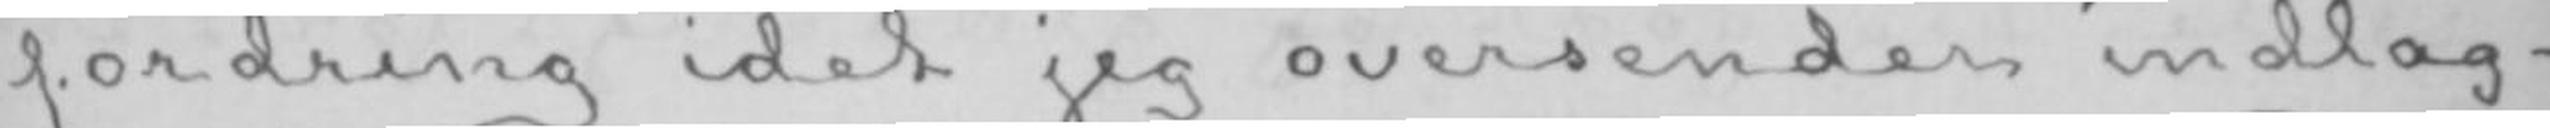fordring idet jeg oversender indlag-
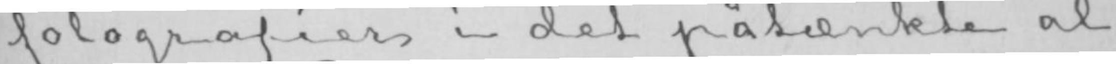fotografier i det påtænkte al-
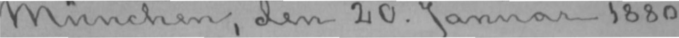München, den 20. Januar 1880.
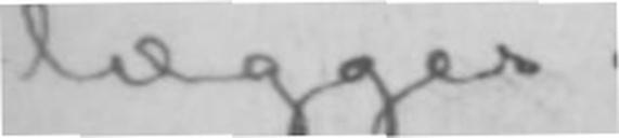lægges.
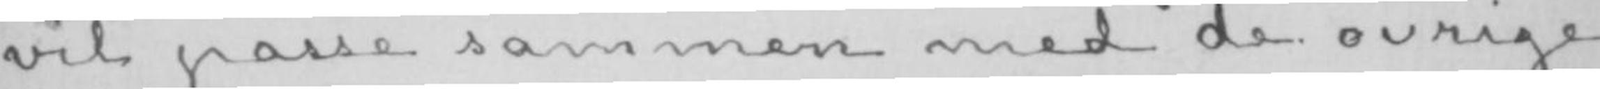vil passe sammen med de øvrige
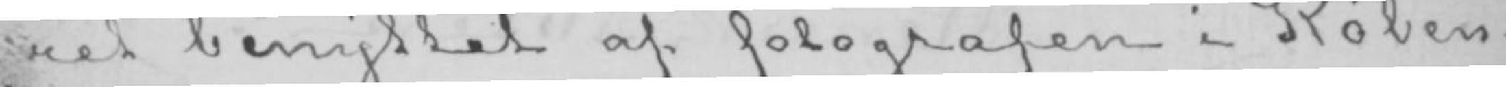ret benyttet af fotografen i Køben-
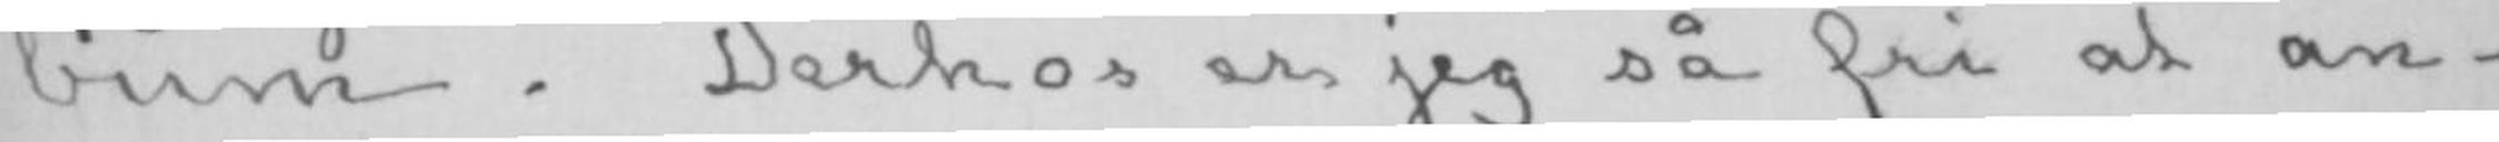bum. Derhos er jeg så fri at an-
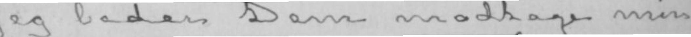Jeg beder Dem modtage min
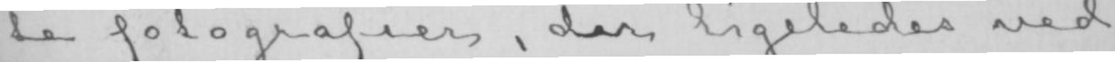te fotografier, der ligeledes ved-
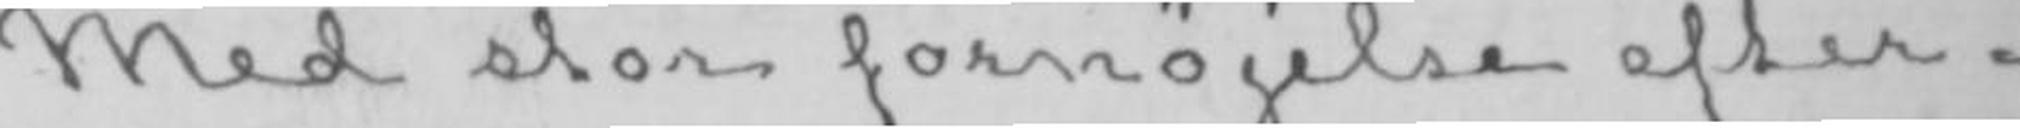Med stor fornøjelse efter-
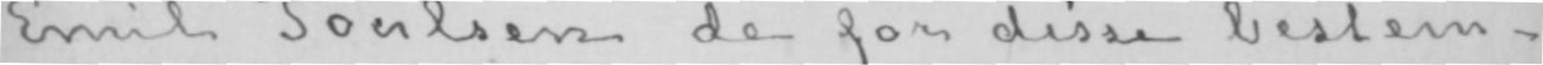Emil Poulsen de for disse bestem-
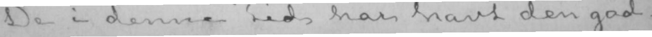De i denne tid har havt den god-
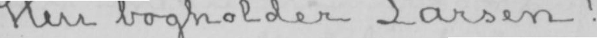Herr bogholder Larsen!
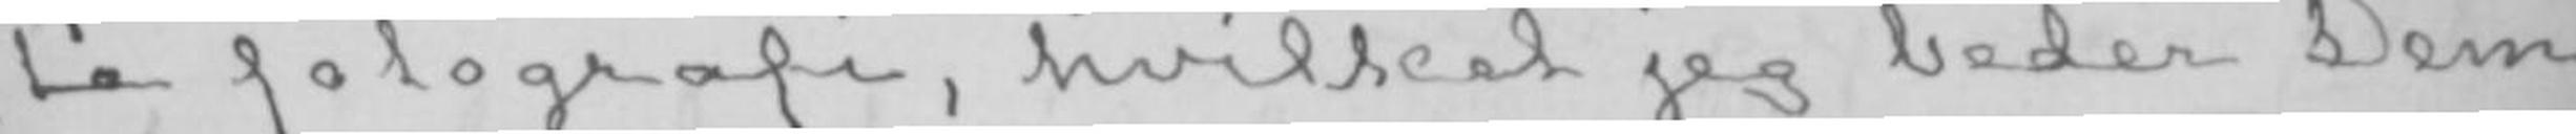te fotografi, hvilket jeg beder Dem
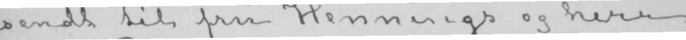sendt til fru Hennings og herr
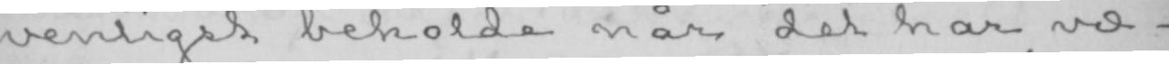venligst beholde når det har væ-
